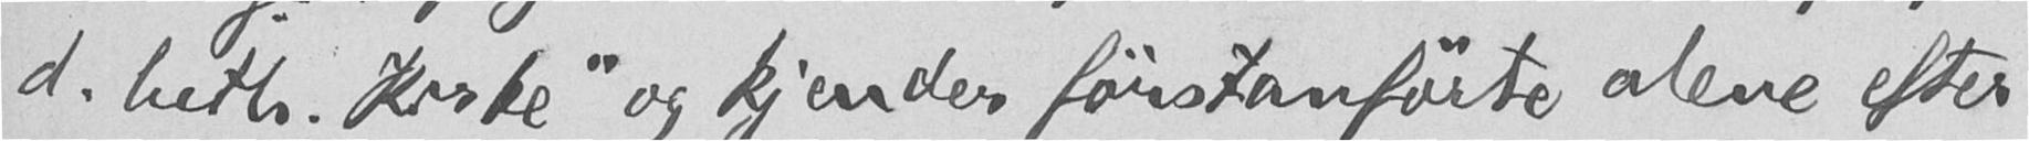d. luth. Kirke" og kjender førstanførte alene efter
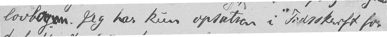lovbog. Jeg har kun opsatsen i "Tidsskrift for
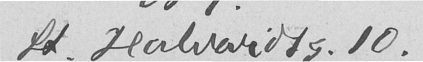St. Halvardsg. 10.
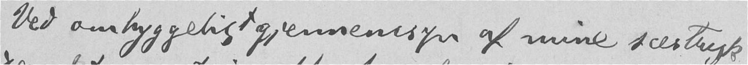Ved omhyggeligt gjennemsyn af mine særtryk
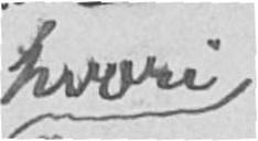hvori
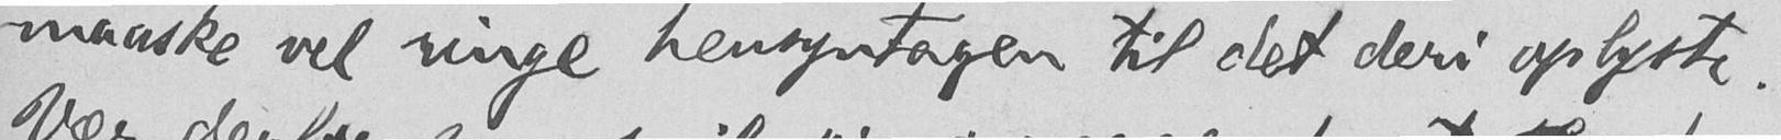maaske vel ringe hensyntagen til det deri oplyste.
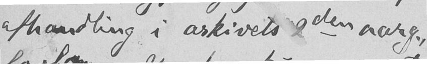afhandling i arkivets 2den aarg.,
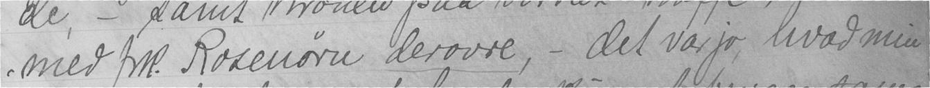med frk. Rosenørn derovre, - det var jo, hvad min
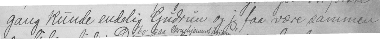gang kunde endelig Gudrun og jeg faa være sammen
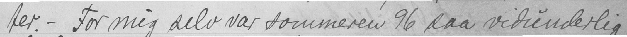ter. - For mig selv var sommeren 96 saa vidunderlig
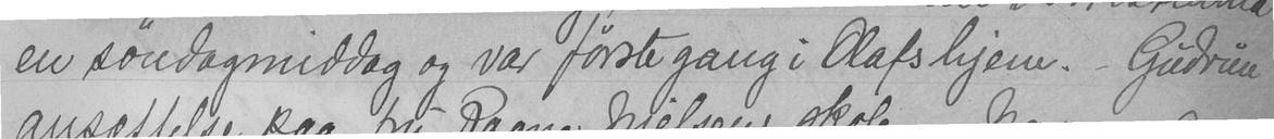en søndagmiddag og var første gang i Oafs hjem. - Gudrun
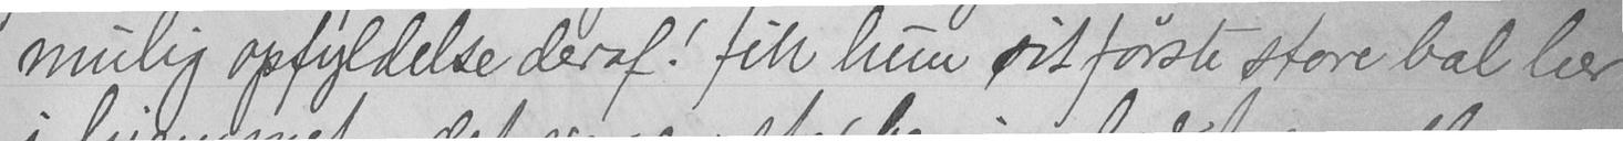mulig opfyldelse deraf! fik hun sit første store bal her
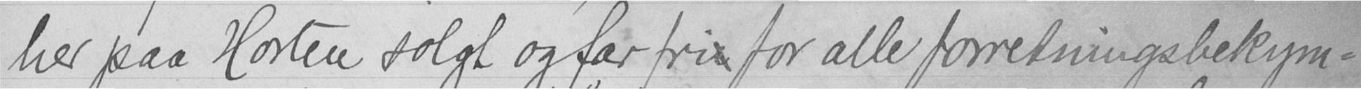her paa Horten solgt og far fri for alle forretningsbekym-
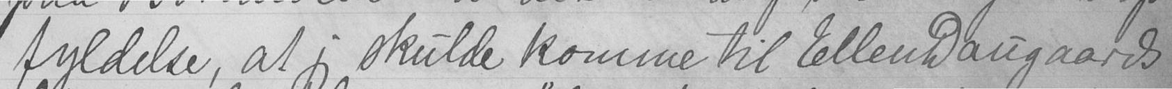fyldelse, at jeg skulde komme til Ellen Daugaards
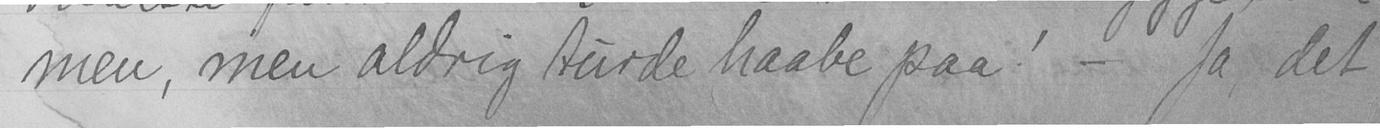men, men aldrig turde haabe paa! - Ja, det
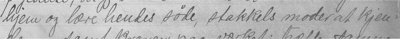hjem og lære hendes søde, stakkels moder at kjen-
